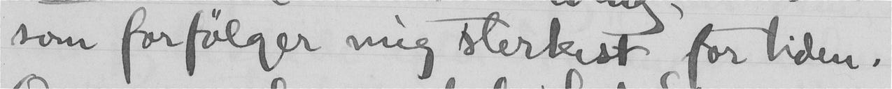som forfølger mig sterkest for tiden.
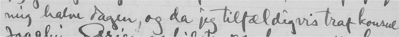mig halve dagen, og da jeg tilfældigvis traf konsul
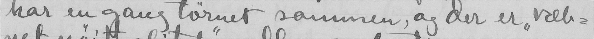har en gang tørnet sammen, og der er "væb-
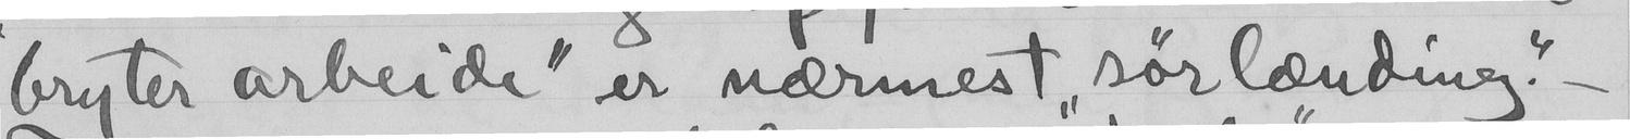bryter arbeide" er nærmest" sørlænding." -
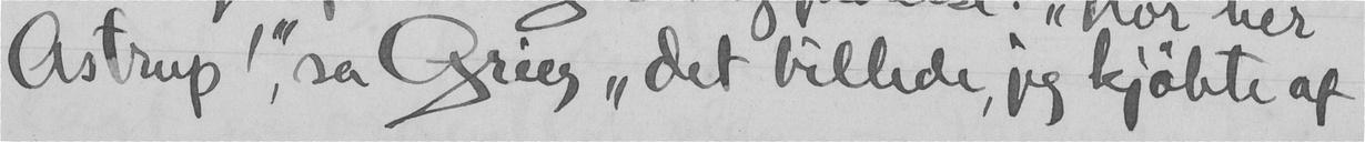Astrup!" sa Greg "det billede, jeg kjøbte af
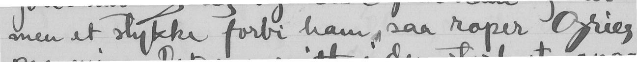men et slykke forbi ham, saa roper Grieg
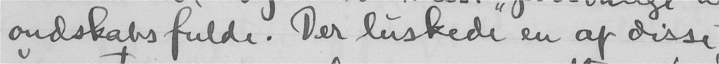ondskabsfulde. Der luskede en af disse
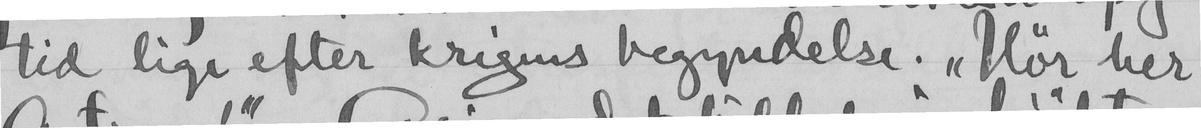tid lige efter krigens begyndelse. "Hør her
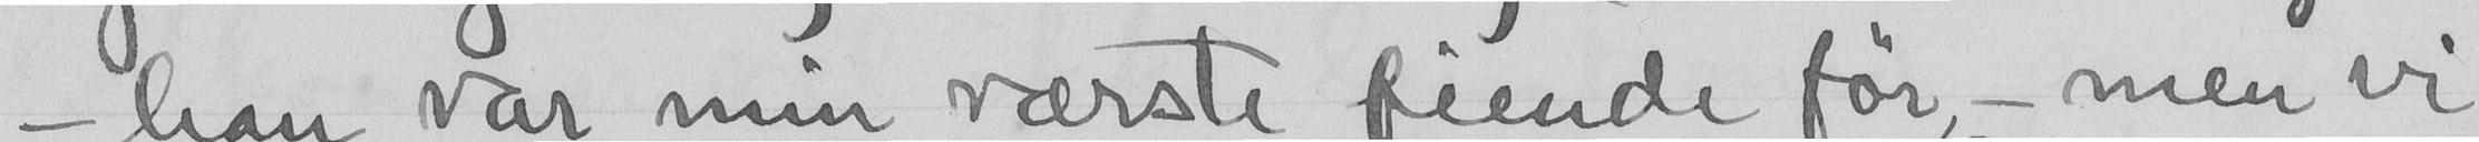- han var min værste fiende før, - men vi
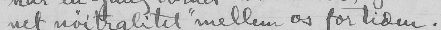net nøitralitet" mellem os for tiden.
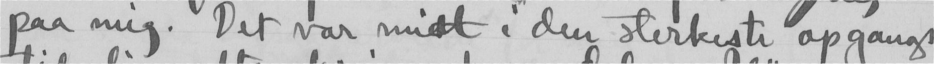paa mig. Det var midt i den sterkeste opgangs
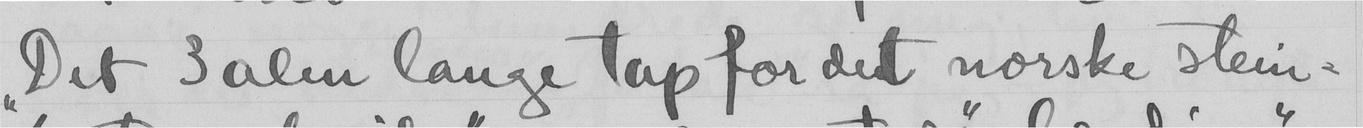"Det 3 alm lange tap for det norske stein-
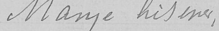Mange hilsener,
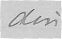din
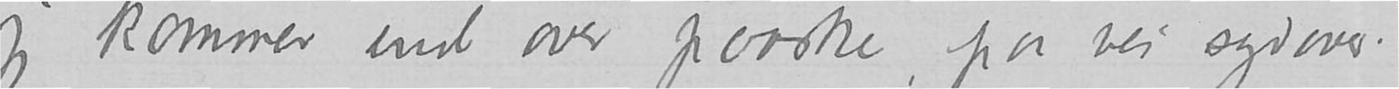jeg kommer ind over paaske, paa vei sydover?
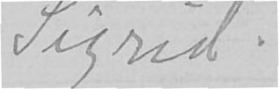Sigrid.
